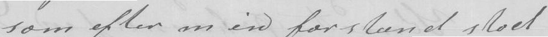som efter min forstand stod
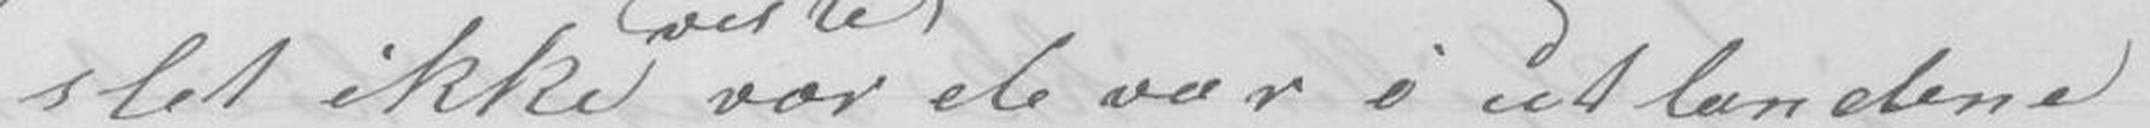slet ikke vor de var i utlandene
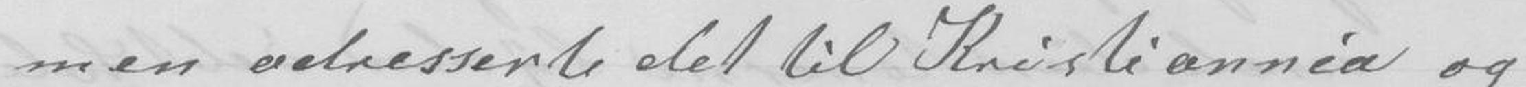men adresserte det til Kristiannia og
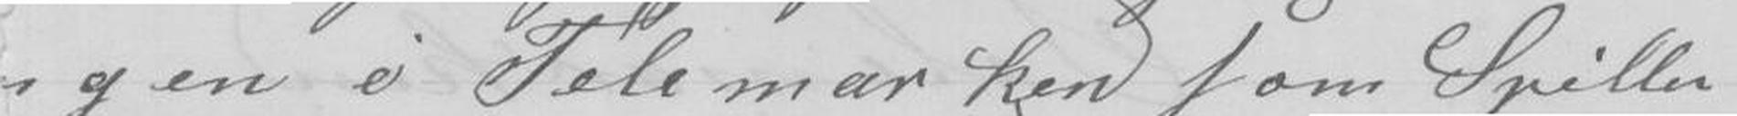jen i Telemarken som Spiller
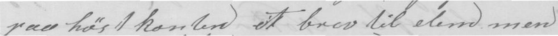paa høstkanten et brev til dem men
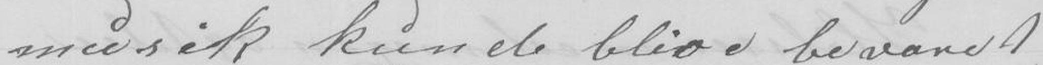musik kunde blive bevaret
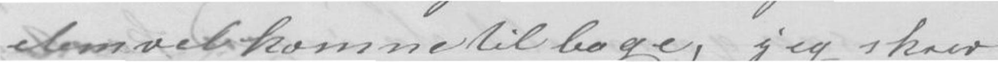dem velkomne tilbage, jeg skrev
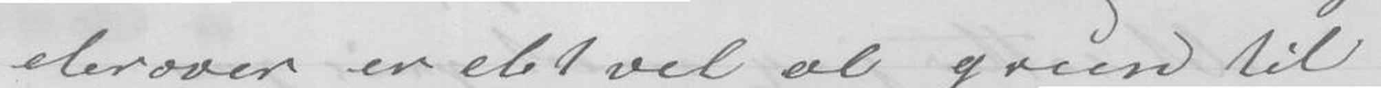derover er det vel al grun til
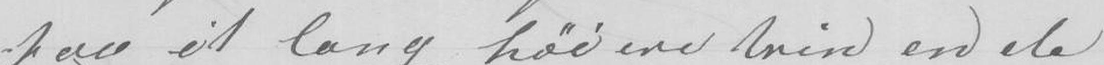paa it lang høiere trin en de
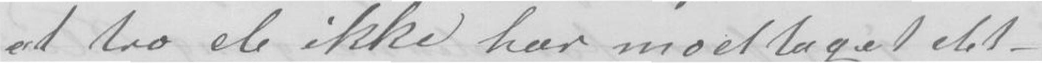at tro de ikke har modtaget det -
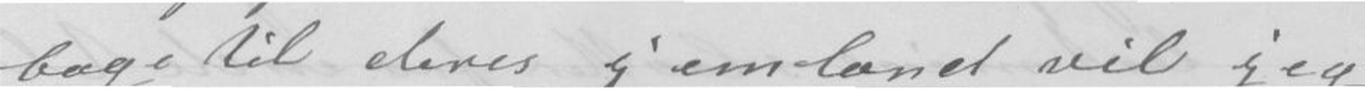bage til deres jemland vil jeg
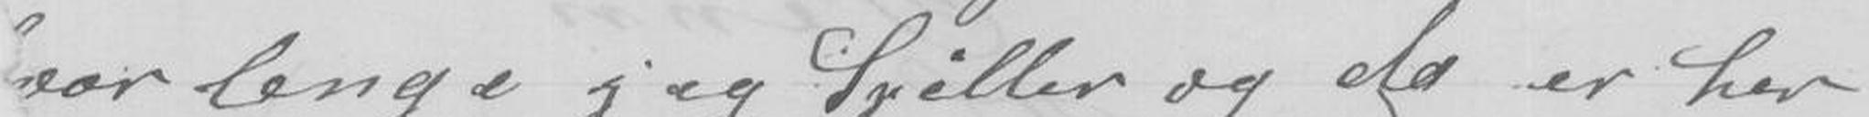vor lenge jeg Spiller og da er her
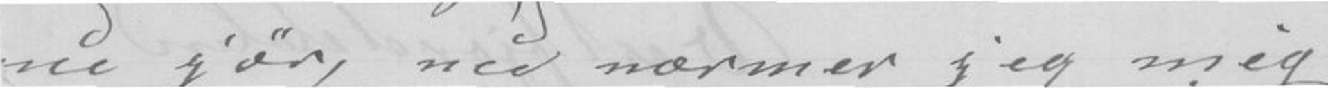nu jør, nu nærmer jeg mig
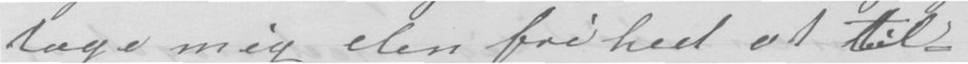tage mig den frihed at til-
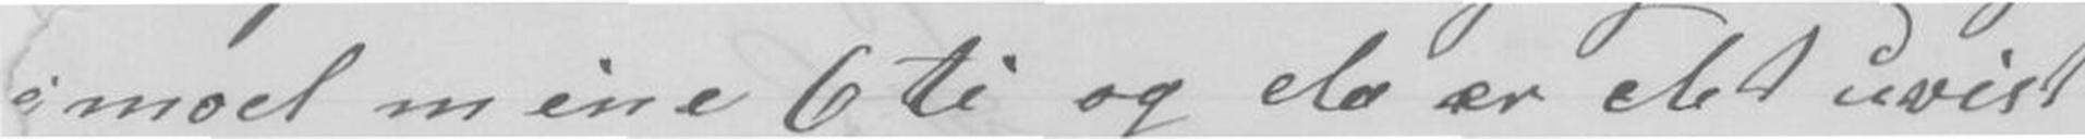imod mine 6 ti og da er det uvist
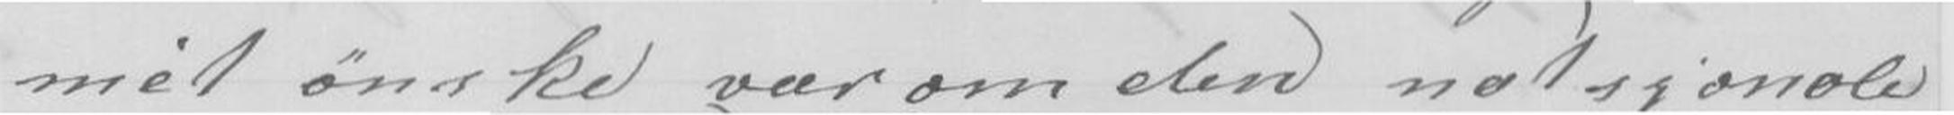mit ønske var om den natsjonale
